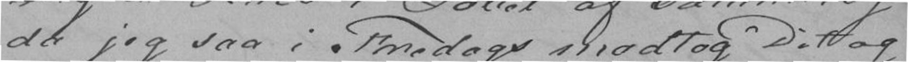da jeg saa i Fredags modtog Dit og
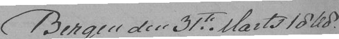Bergen den 31te Marts 1868.
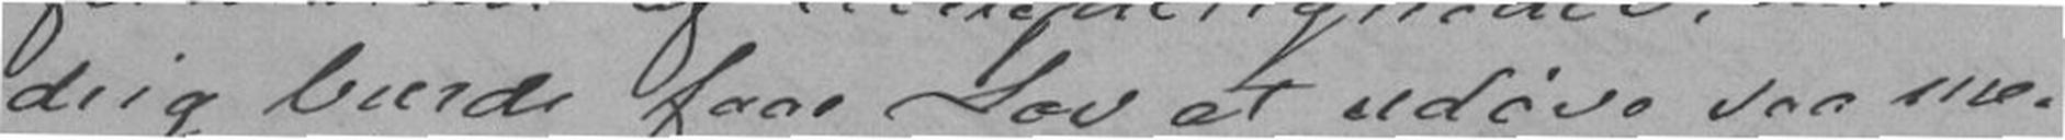drig burde faae Lov at udøve saa me-
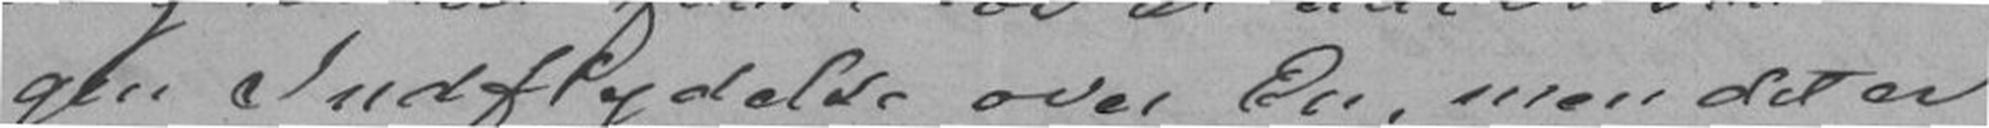gen Indflydelse over En, men det er
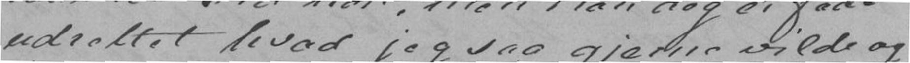udrettet hvad jeg saa gjerne vilde og
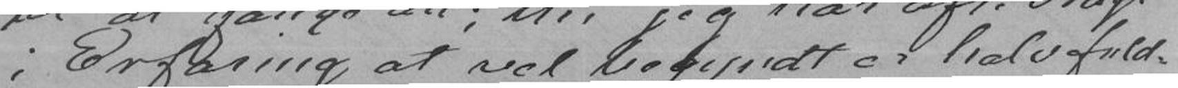i Erfaring at vel begyndt er halvfuld-
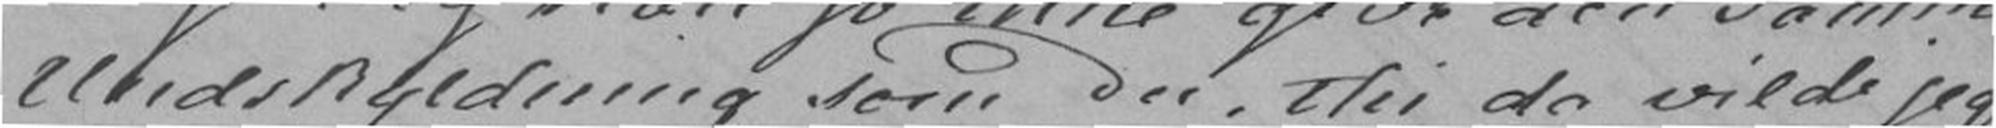Undskyldning som Du, thi da vilde jeg
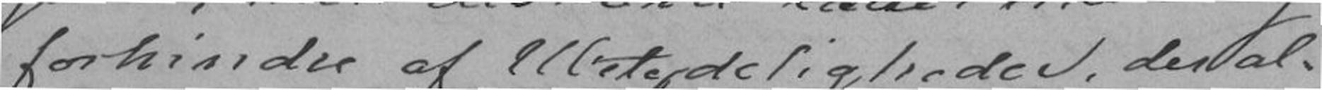forhindre af Ubetydeligheder, der al-
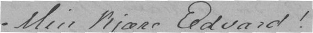Min kjære Edvard!
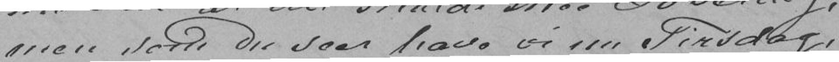men som Du seer have vi nu Tirsdag,
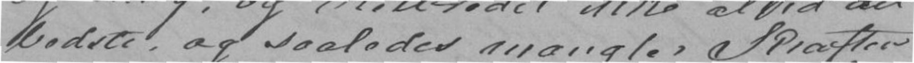bedste, og saaledes mangler Kraften
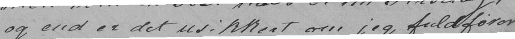og end er det usikkert om jeg fuldfører
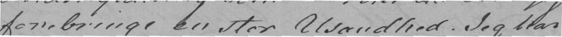forebringe en stor Usandhed. Jeg har
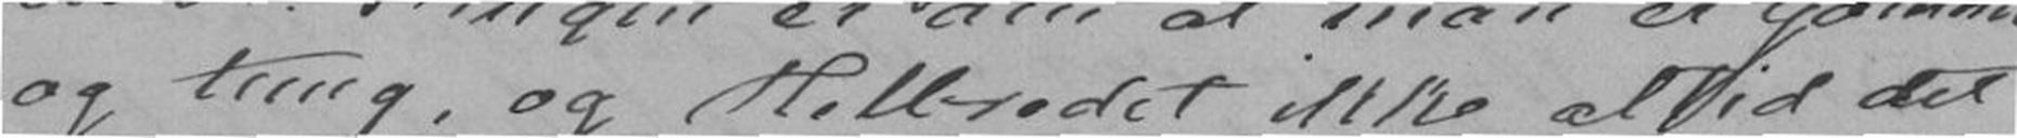og tung, og Helbredet ikke altid det
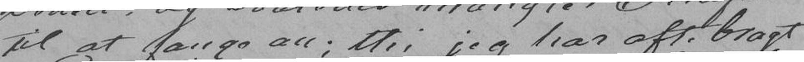til at fange an; thi jeg har ofte bragt
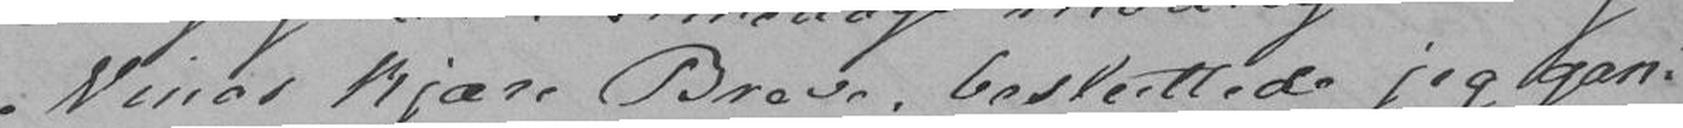Ninas kjære Breve, besluttede jeg gan-
